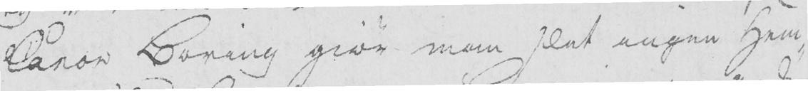Canon Boring giør man slet ingen Hem-
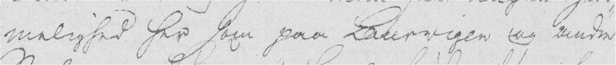melighed her som paa Laurvigen og andre
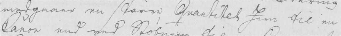medgaaer en større Quantitet Jern til en
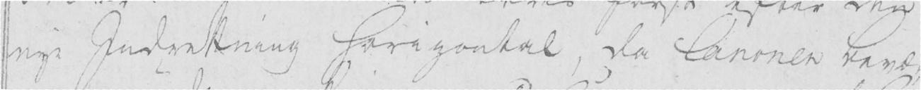nye Indrettning horizontal, da Canonen bevæ-
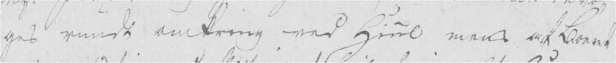ges rundt omkring ved Hiul mens af Borret
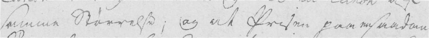samme Størrelse; og at Prisen paa en saadan
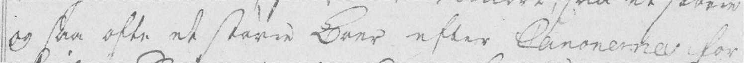og saa ofte et større Boer efter Canonernes for-
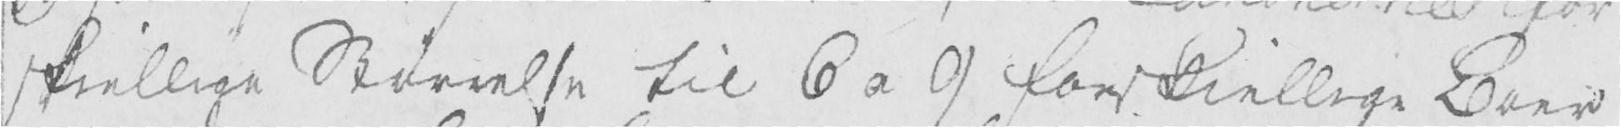skiellige Størrelse til 6 a 9 forskiellige Boer
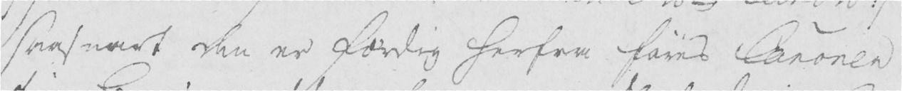saasnart den er færdig herfra føres Canonen
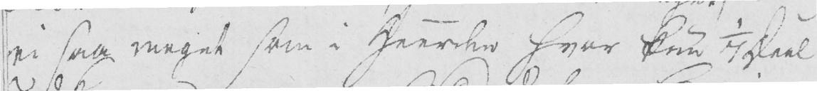er saa meget som i Heerden hvor kun 1/7 Deel
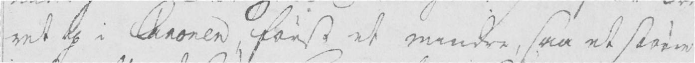ret op i Canonen, først et mindre, saa et større
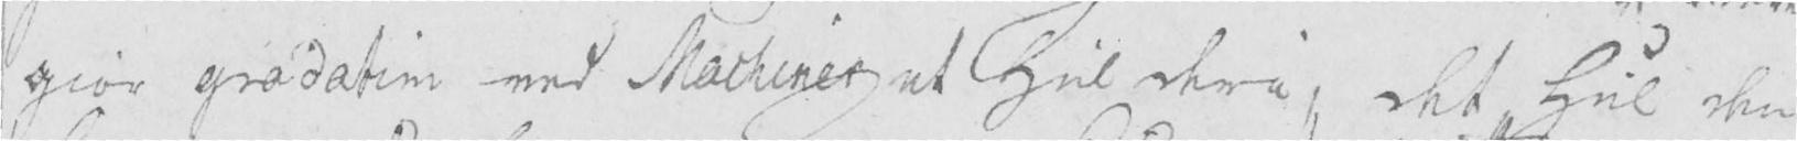giør gradatim ved Machiner et Hul deri, det Hul den
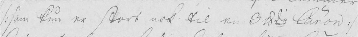/: som kun er stort nok til en 3 sdg Canon :/
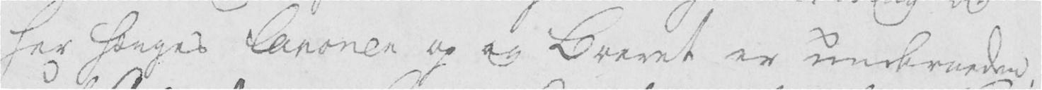her hænges Canonen op og Boeret er underneden,
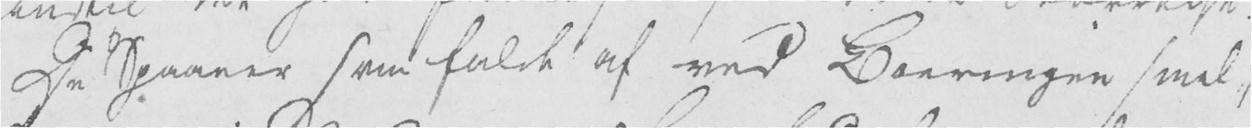De Spaaner som falde af ved Boeringen smel-
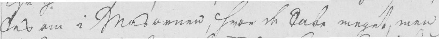tes om i Masovnen, hvor de tabe meget, men
